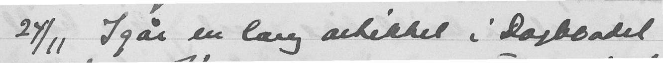27/11 Igår en lang artikkel i Dagbladet
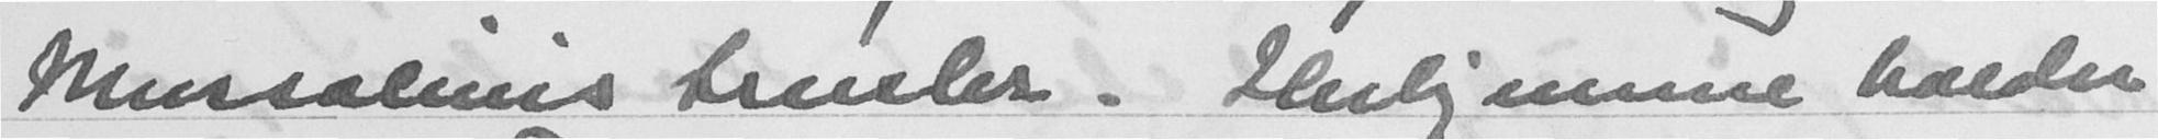Mussolinis trusler. Herhjemme holder
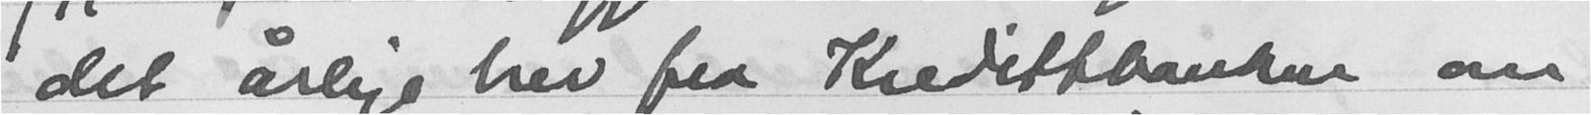det årlige brev fra Kredittbanken om
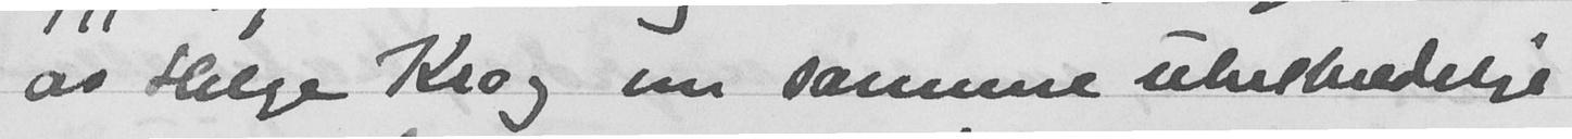av Helge Krog om samme uhelbredelige
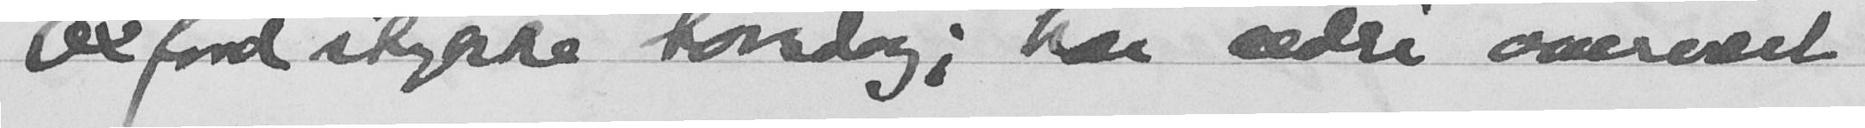Oxfordstykke torsdag; har aldri overvært
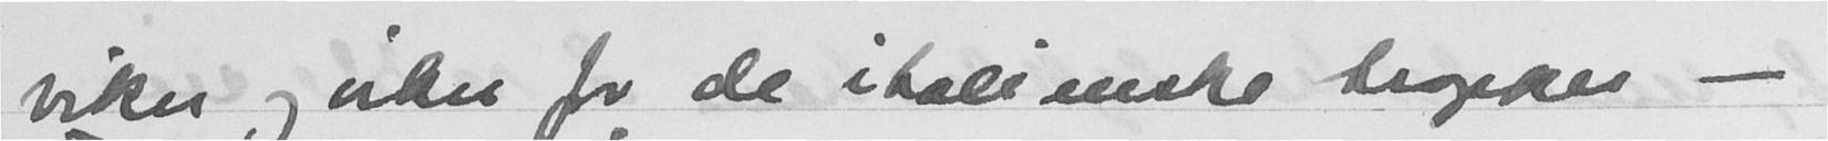viker og virker for de italienske tropper -
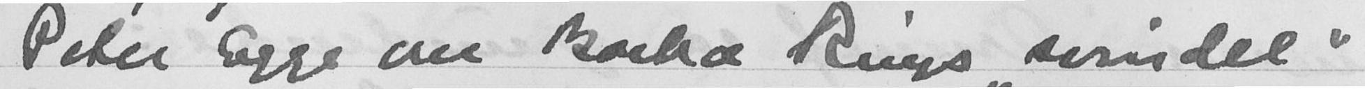Peter Egge om Barbra Rings "svindel"
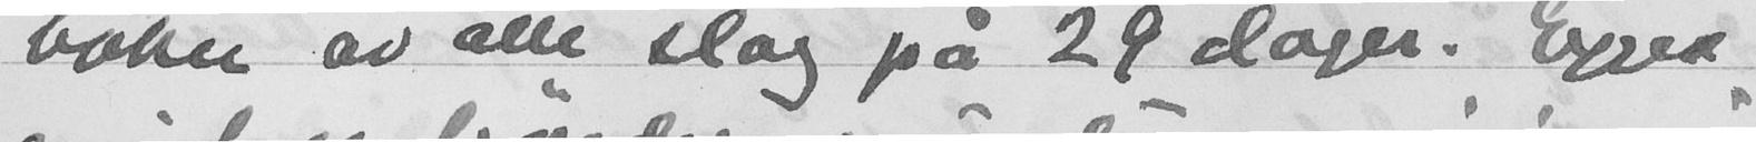bober av alle slag på 28 dager. Egge
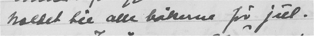holdet til alle bøkerne før jul.
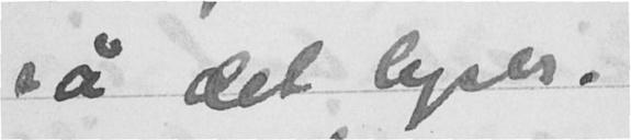så det lyser.
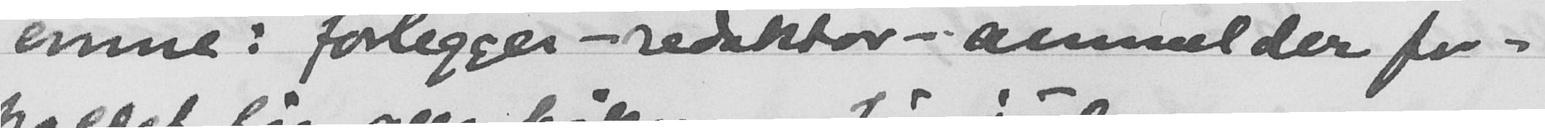emne: forlegger-redaktør-ammelder for-
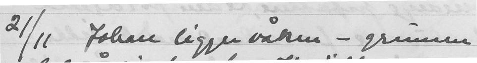2/111 Johan ligger våken - grunner
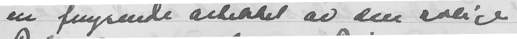en fnysende artikkel av den salige
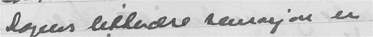Dagens litteære semaajon er
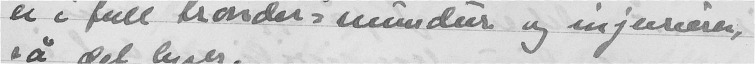er i full trønder-mundur og ijenierer,
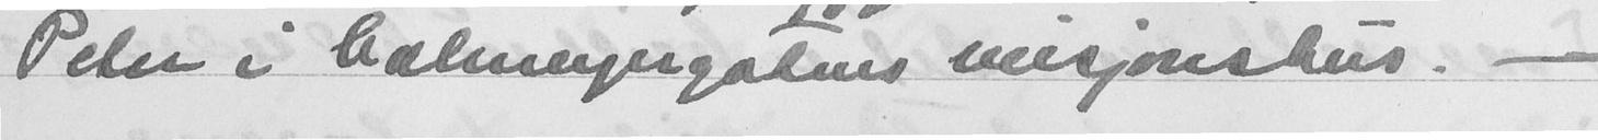Peter i Calmyngadens misjonshus -
